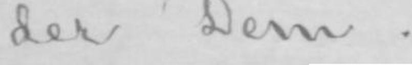der Dem.
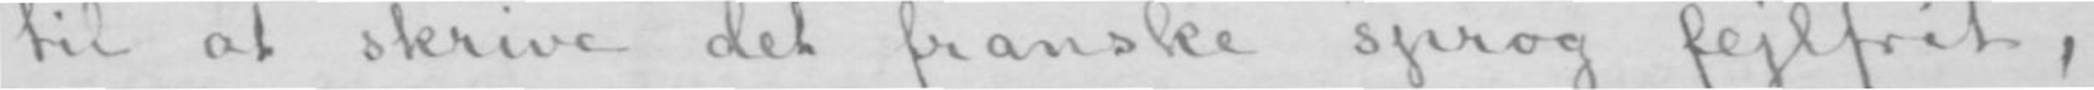til at skrive det franske sprog fejlfrit,
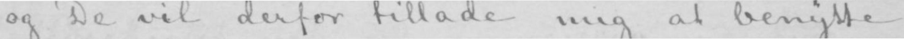og De vil derfor tillade mig at benytte
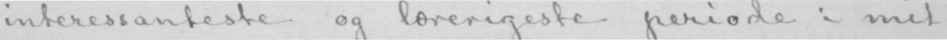interessanteste og lærerigeste periode i mit
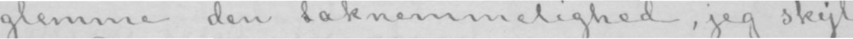glemme den taknemmelighed, jeg skyl-
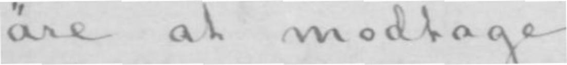äre at modtage.
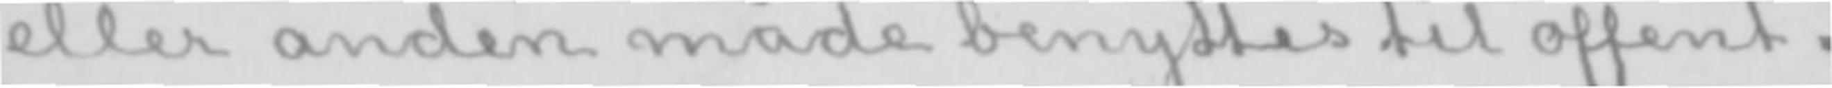eller anden måde benyttes til offent-
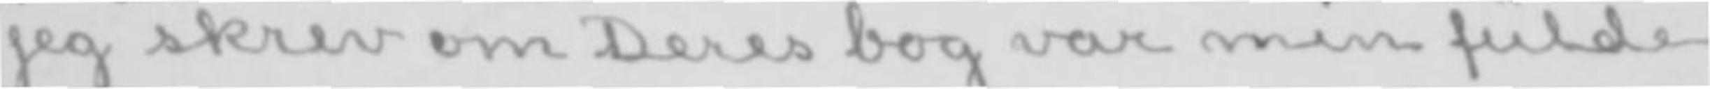jeg skrev om Deres bog var min fulde
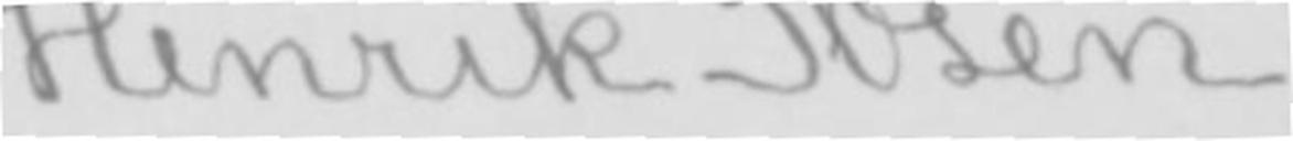Henrik Ibsen.
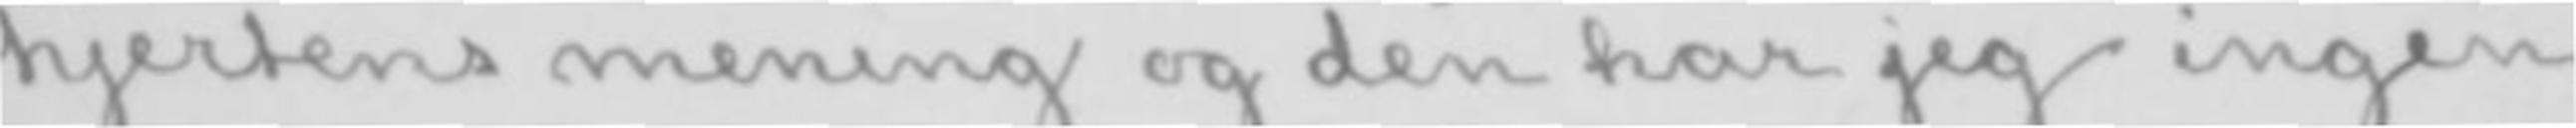hjertens mening og den har jeg ingen
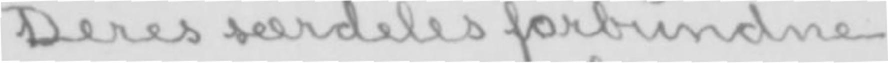Deres særdeles forbundne
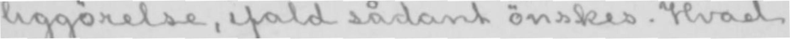liggørelse, ifald sådant ønskes. Hvad
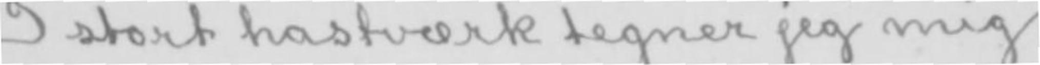I stort hastværk tegner jeg mig
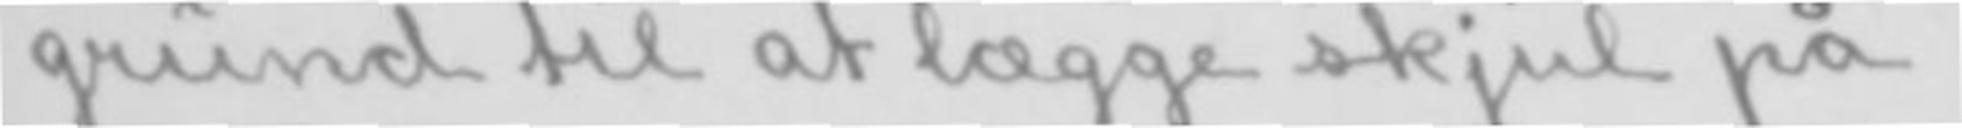grund til at lægge skjul på.
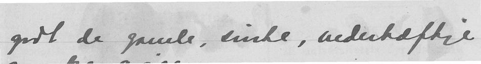godt de gamle, sinte, vederhæftige
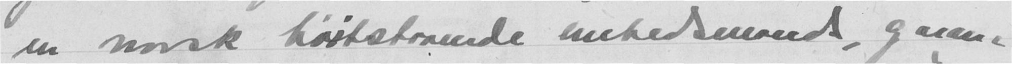en norsk høitstaaende embedsmand, garan-
